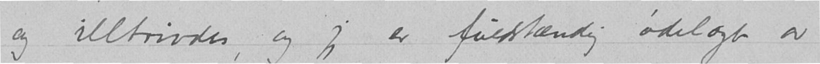og illtrivdes, og jeg er fuldstændig ødelagt av
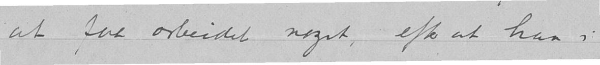at faa arbeidet noget, efter at han i
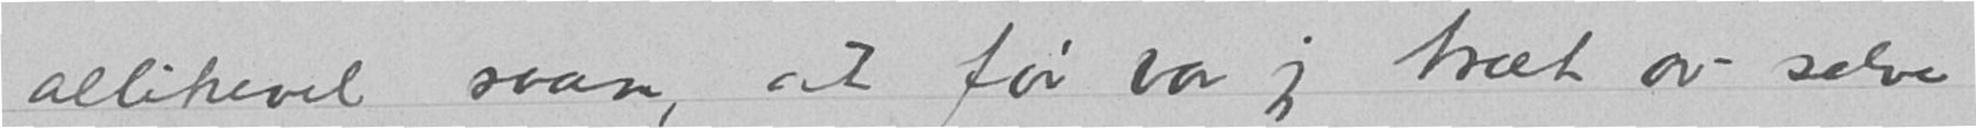allikevel saan, at før var jeg træt av selve
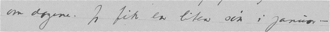om dagene. Jeg fik en liten søn i januar -
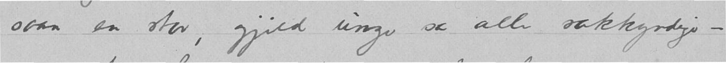saan en stor, gjild unge sa alle sakkyndige -
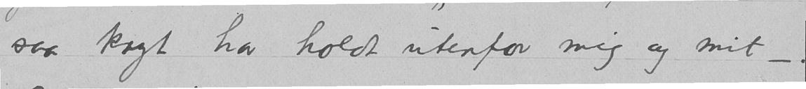saa kægt har holdt utenfor mig og mit.
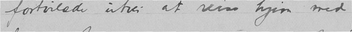fortvilede utvei at reise hjem med
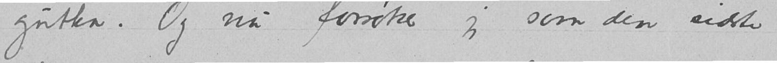gutten. Og nu forsøker jeg som den sidste
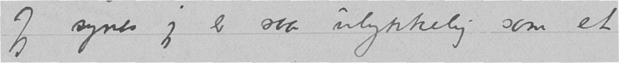Jeg synes jeg er saa ulykkelig som et
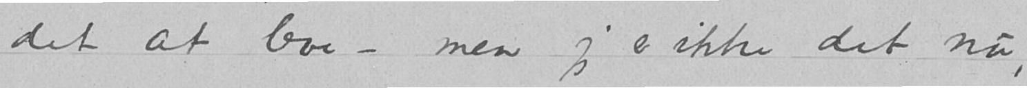det at leve - men jeg er ikke det nu,
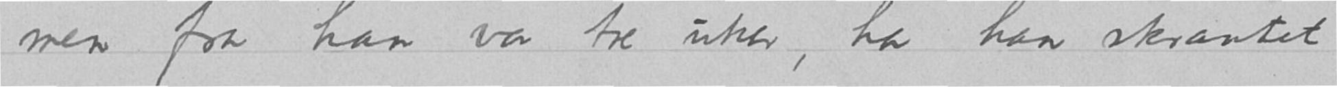men fra han var tre uker, har han skrantet
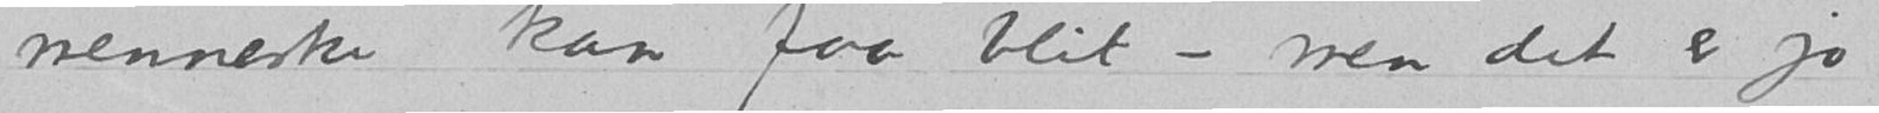menneske kan faa blit – men det er jo
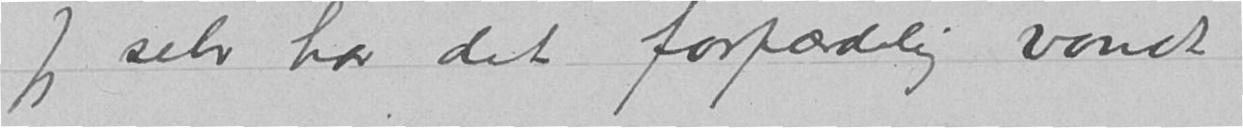Jeg selv har det forfærdelig vondt
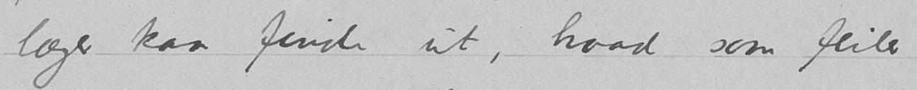læger kan finde ut, hvad som feiler
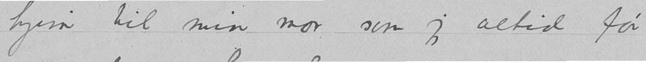hjem til min mor som jeg altid før
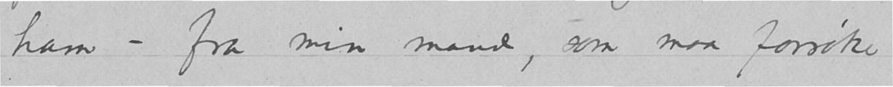ham – fra min mand, som maa forsøke
